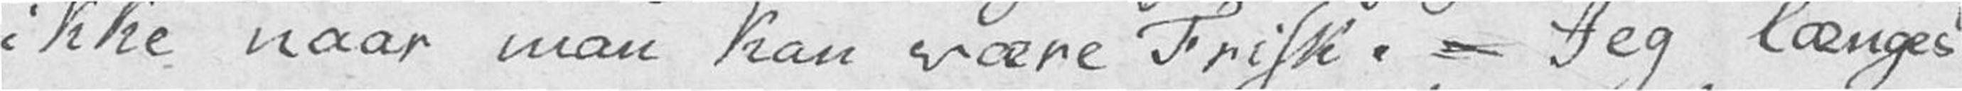ikke naar man kan være Frisk. – Jeg længes
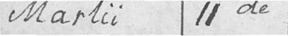Martii 11de
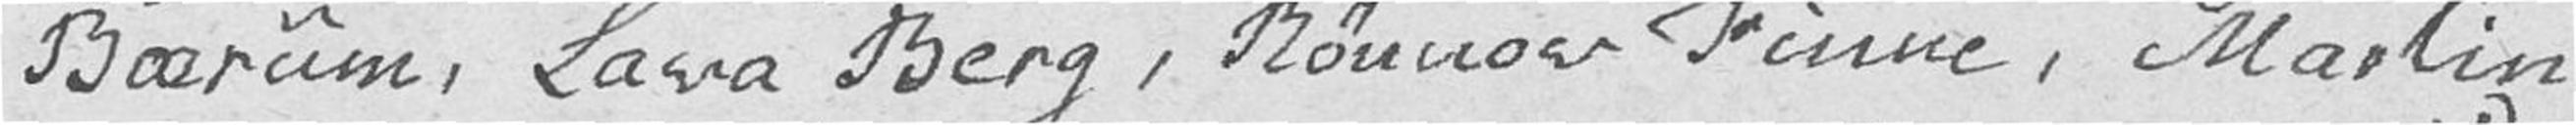Bærum, Lava Berg, Rønnov Finne, Martin
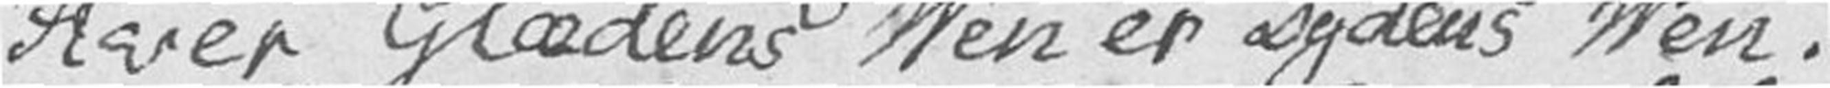Hver Glædens Ven er Dydens Ven.
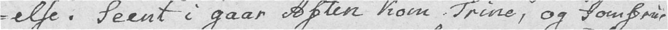-else. Seent i gaar Aften kom Trine, og Jomfru
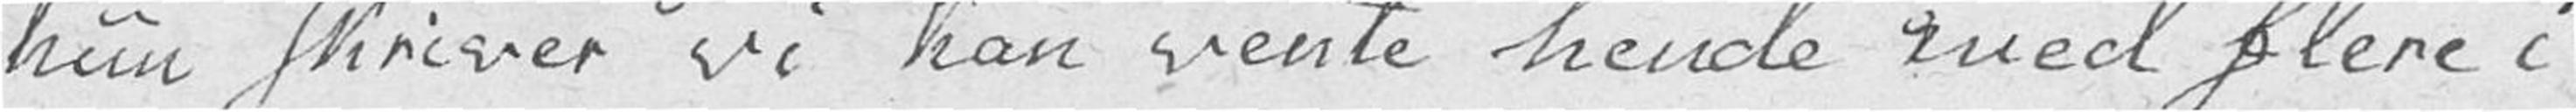hun skriver vi kan vente hende med flere i
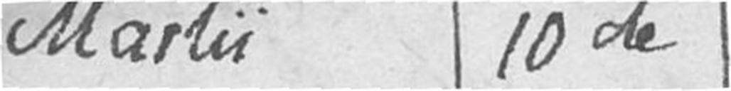Martii 10de
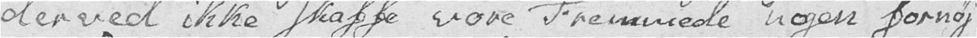derved ikke skaffe vore Fremmede nogen fornøj
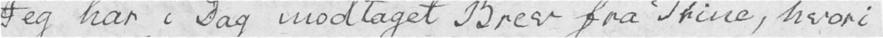Jeg har i Dag modtaget Brev fra Trine, hvori
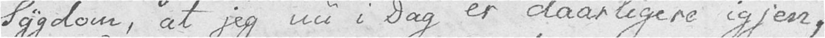Sygdom, at jeg nu i Dag er daarligere igjen,
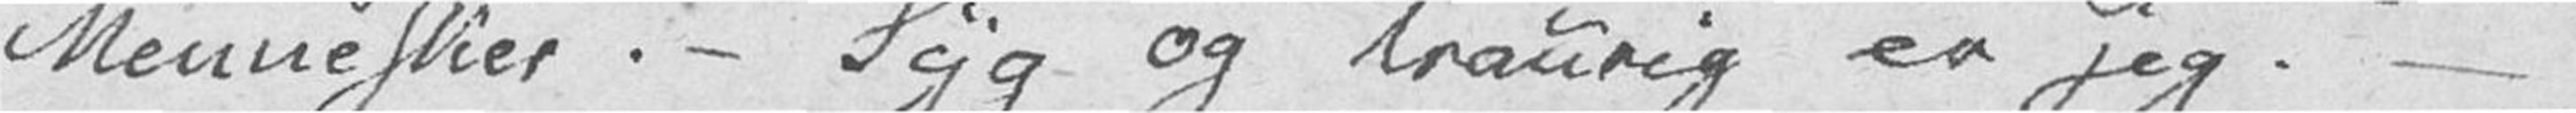Mennesker. – Syg og traurig er jeg. –
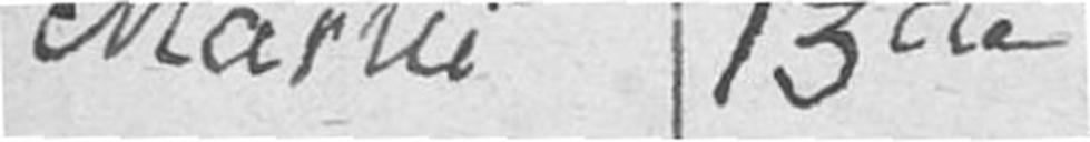Martii 13de
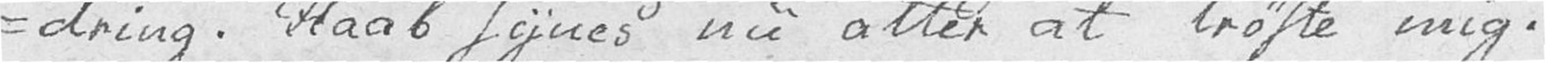-dring. Haab synes nu atter at Trøste mig.
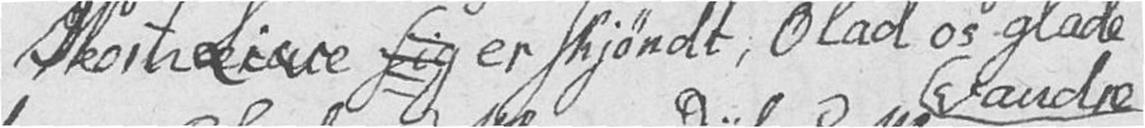Vort Liv sig er skjøndt, O lad os glade vandre
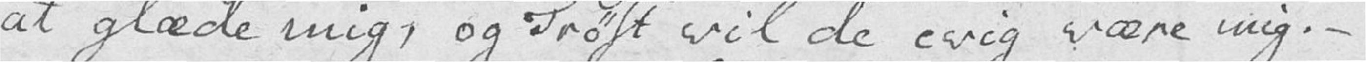at glæde mig, og Trøst vil de evig være mig. –
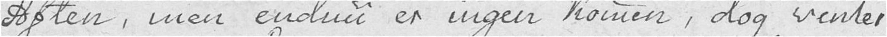Aften, men endnu er ingen kommen, dog venter
